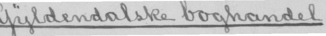Gyldendalske boghandel,
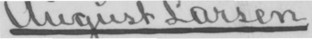August Larsen,
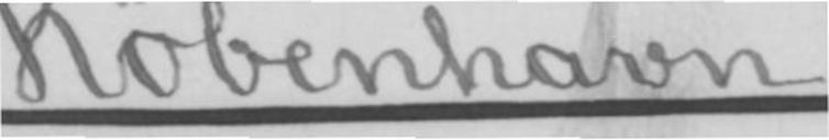København.
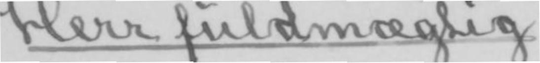Herr fuldmægtig
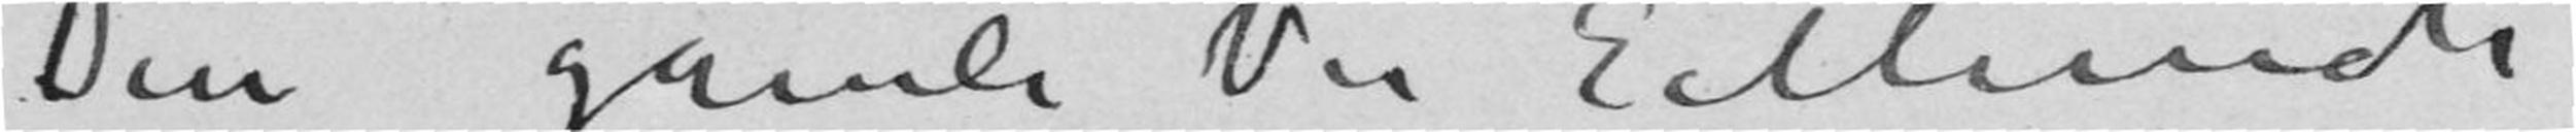Din gamle Ven E Munch
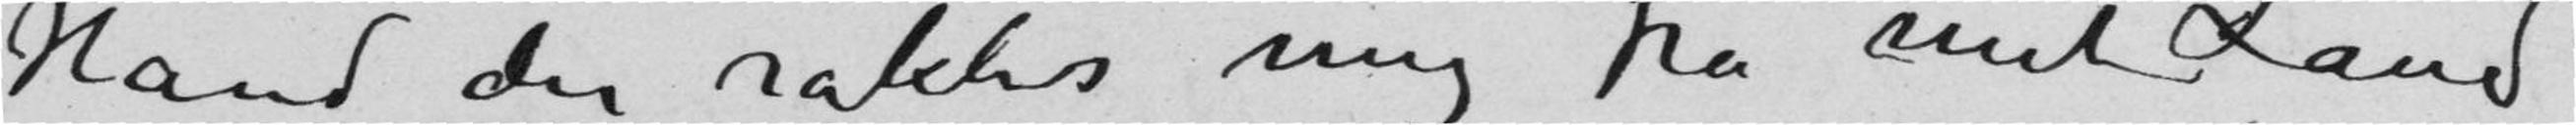Hånd der raktes mig fra mit Land
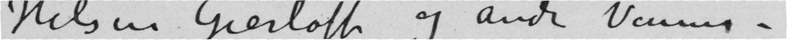Hilser Gierløff og andre Venner –
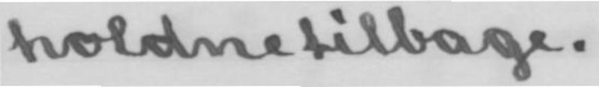holdne tilbage.
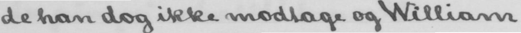de han dog ikke modtage og William
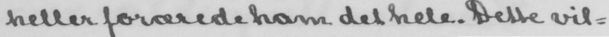heller forærede ham det hele. Dette vil-
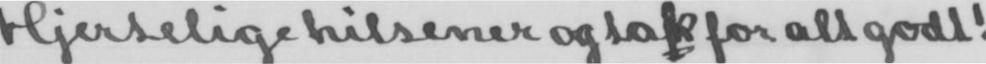Hjertelige hilsener og tak for alt godt!
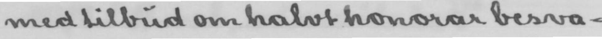med tilbud om halvt honorar besva-
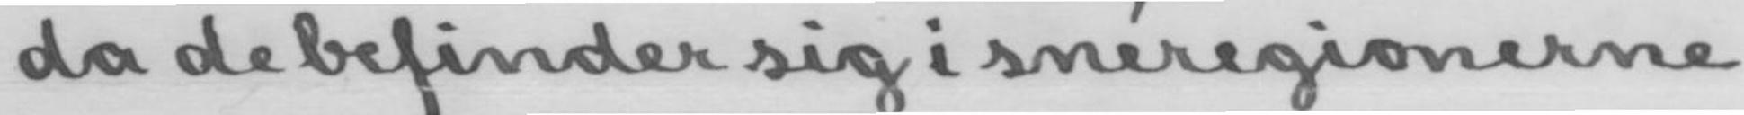da de befinder sig i snéregionerne
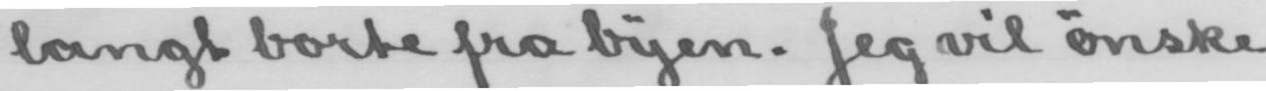langt borte fra byen. Jeg vil ønske
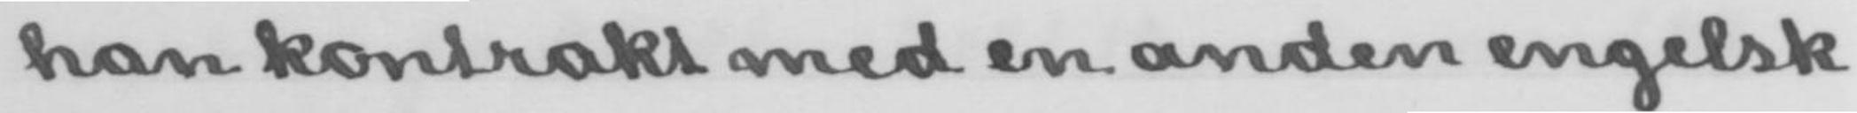han kontrakt med en anden engelsk
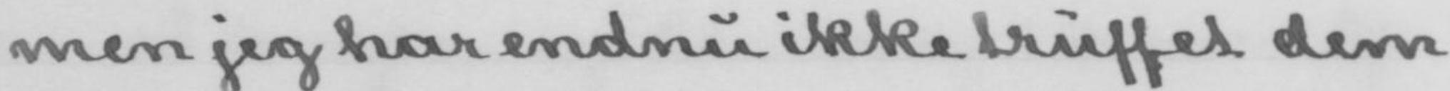men jeg har endnu ikke truffet dem
